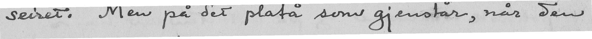seiret. Men på det platå som gjenstår, når den
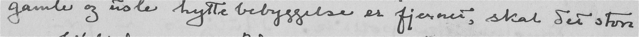gamle og usle hyttebebyggelse er fjernet, skal det store
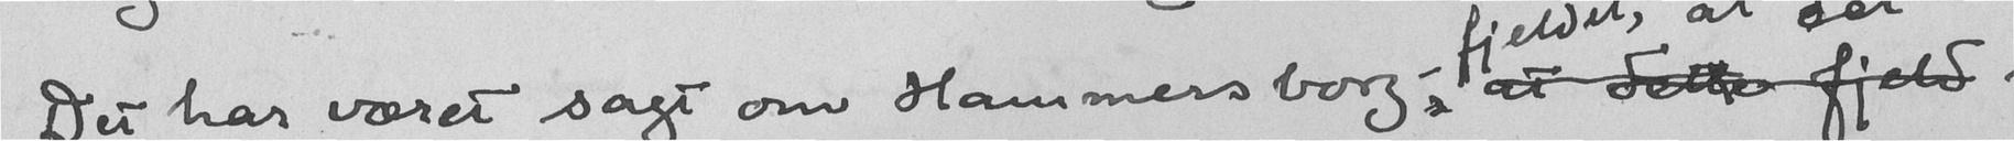Det har været sagt om Hammersborg, at dette fjeld
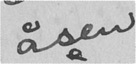åsen
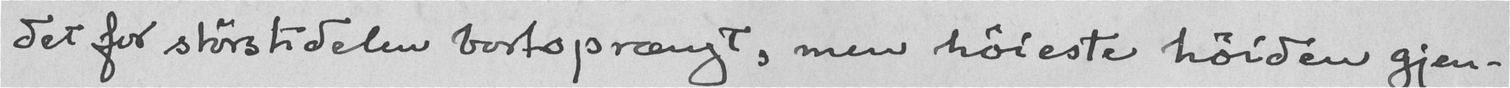det for størstidelen bortsprængt, men høieste høiden gjen-
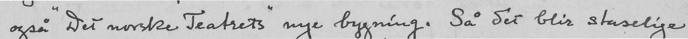også "Det norske Teatrets" nye bygning. Så det blir staselige
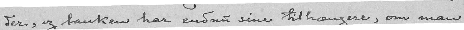der, og tanken har endnu sine tilhængere, om man
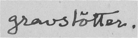gravstøtter.
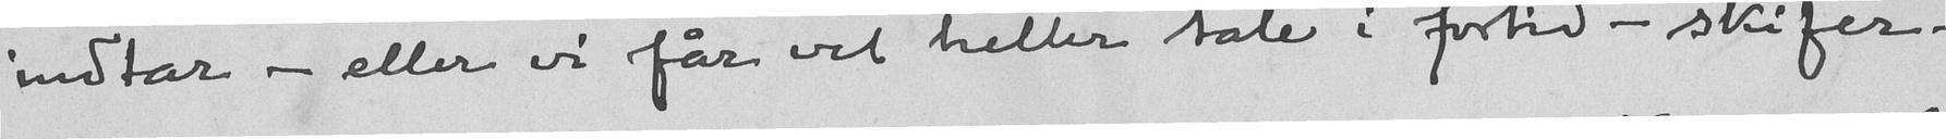indtar - eller vi får vel heller tale i fortid - skifer-
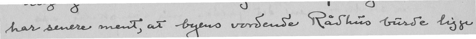har senere ment, at byens vordende Rådhus burde ligge
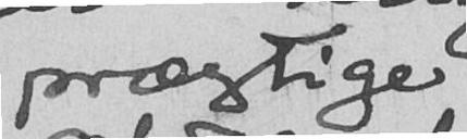prægtige
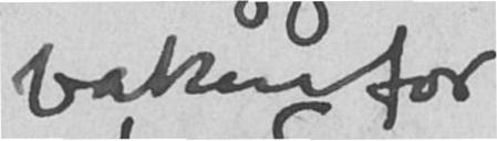bakenfor
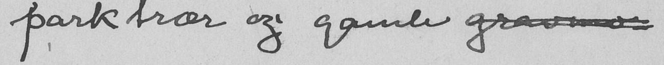parktrær og gamle gravene
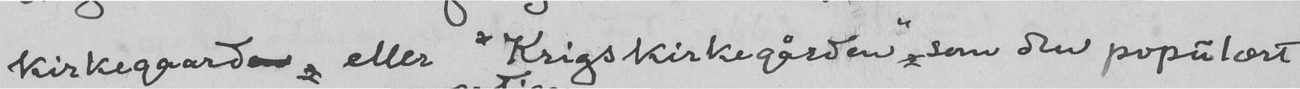kirkegaarden, eller "Krigskirkegården", som den populært
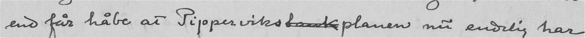end får håbe at Pippervikstankplanen nu endelig har
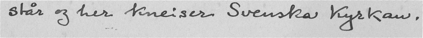står og her kneiser Svenska Kyrkan.
